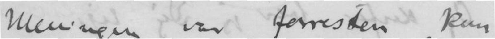Meningen var forresten kun
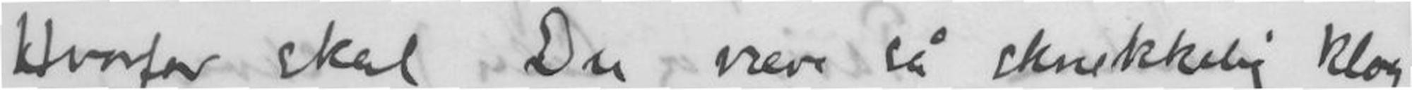Hvorfor skal Du være så skrukkelig klog,
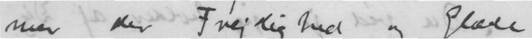mer der Frejdighed og Glæde
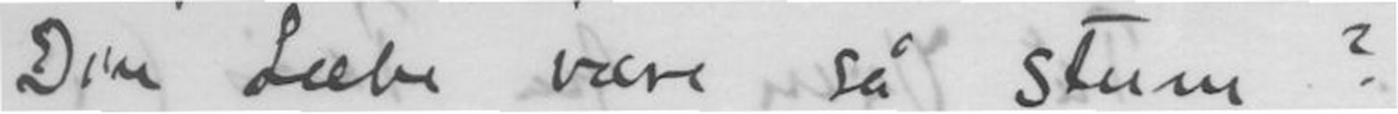Din Læbe være så stum?
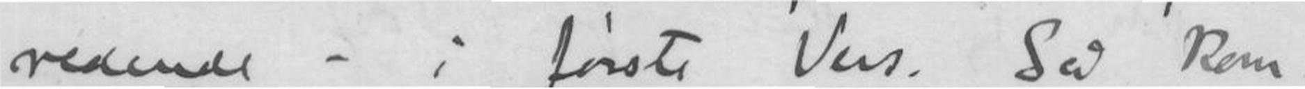redende - i første Vers. Så kom
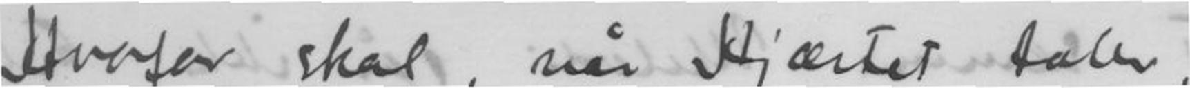Hvorfor skal, når Hjærtet taler,
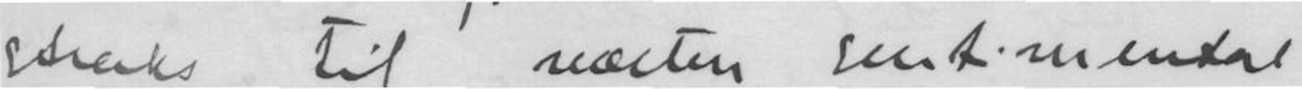straks til næsten sentimental
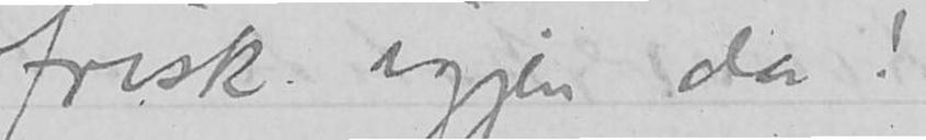frisk igjen da!
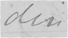din
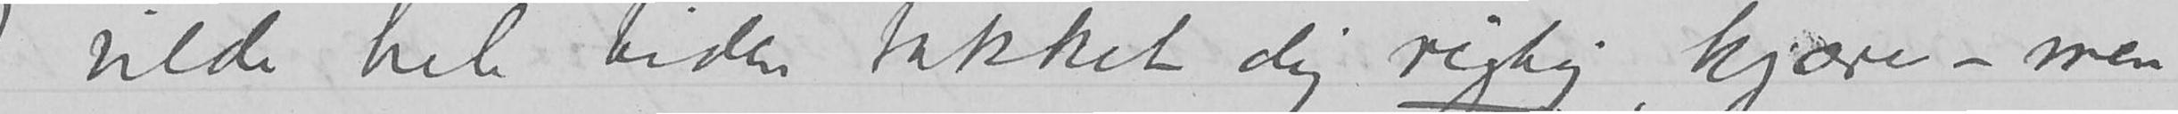- Jeg vilde hele tiden takket dig rigtig , kjære – men
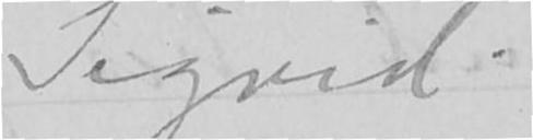Sigrid
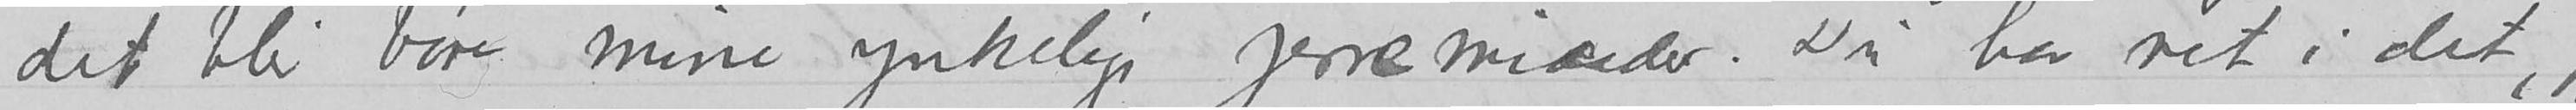det blir bare mine ynkelige jeremiader. Du har ret i det,
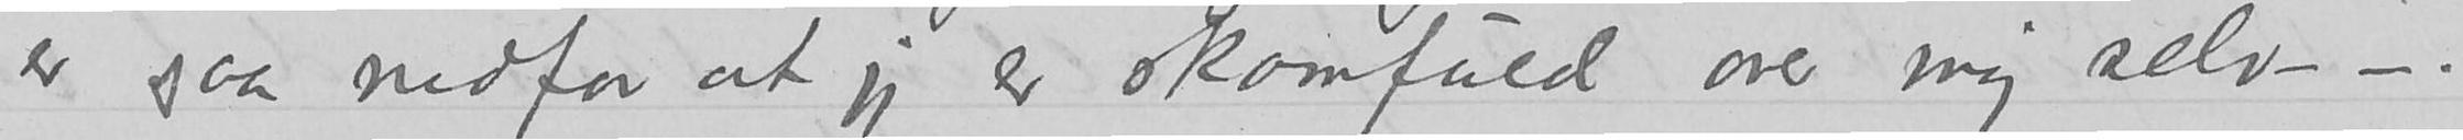jeg er saa nedfor at jeg er skamfuld over mig selv - -.
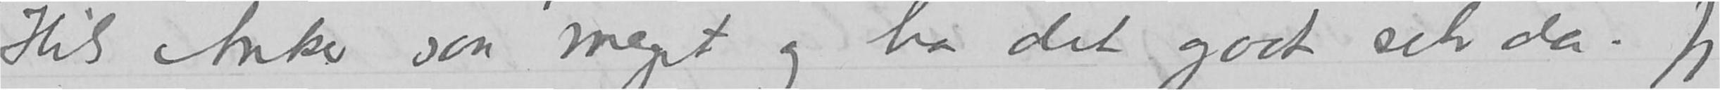Hils Anker saa meget og ha det godt selv da. Jeg
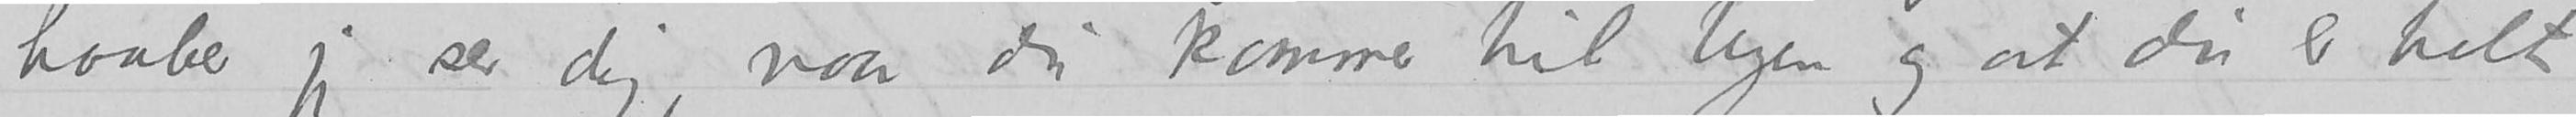haaber jeg ser dig, naar du kommer til byen og at du er helt frisk igjen da!
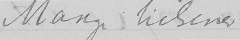Mange hilsener
